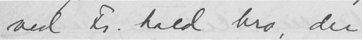ved Fr. hald bro, der
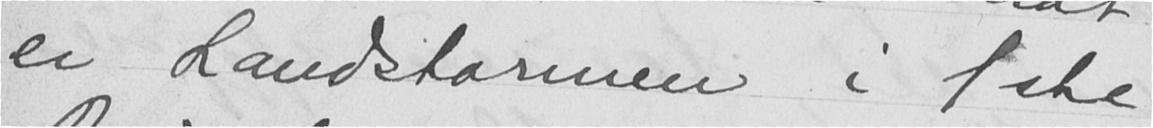er Landstormen i 1ste
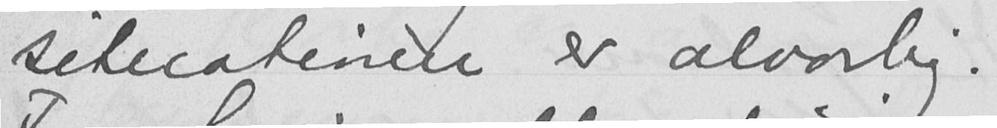situationen er alvorlig.
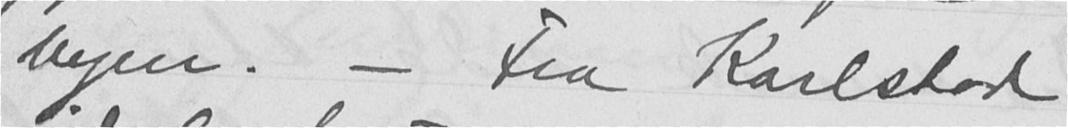byen. - Fra Karlstad
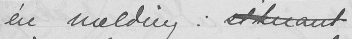én melding: stituant
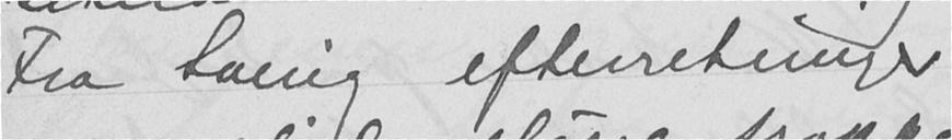Fra Sverig efterretninger
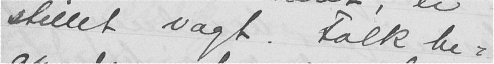stillet vagt. Folk be-
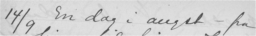14/9 En dag i angst - fra
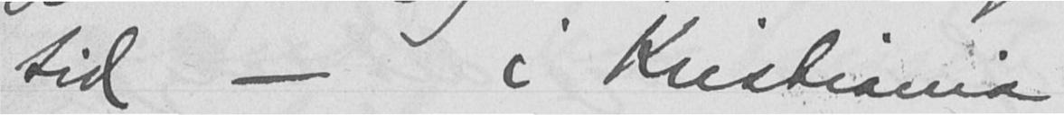tid - i Kristiania
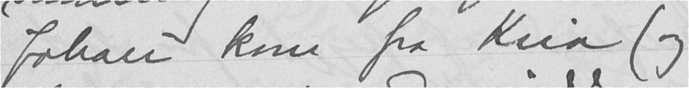Johan kom fra Kria (og
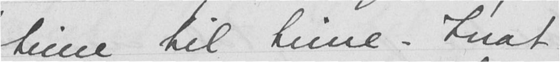time til time. Inat
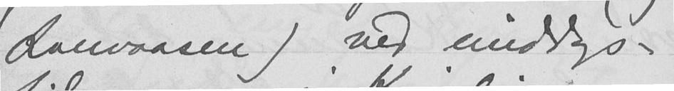Lauvaasen) ved middags-
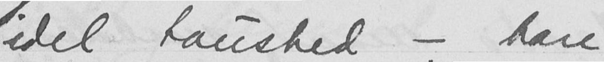idel taushed - bare
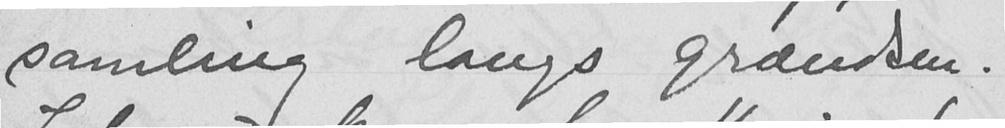samling langs grændsen.
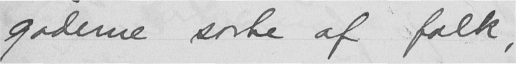gaderne sorte af folk,
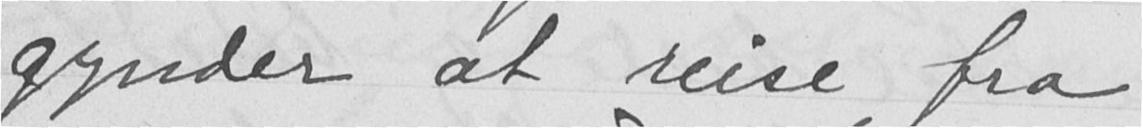gynder at reise fra
